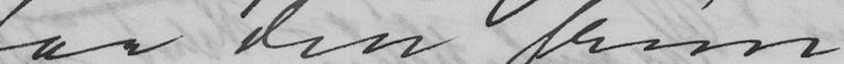faa den frem.
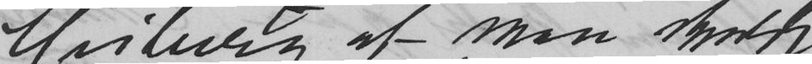Heiberg at man skulde
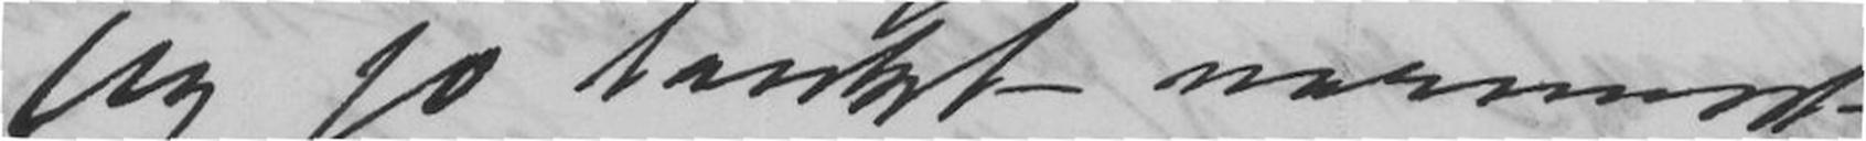jeg jo tænkt nærmest
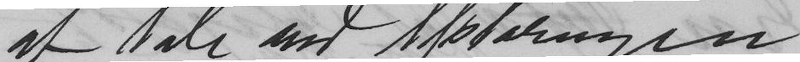at tale ved Afsløringen
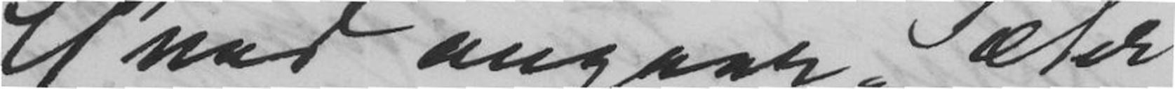Hvad angaar Sæter
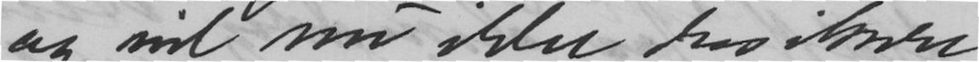og vil nu ikke resikere
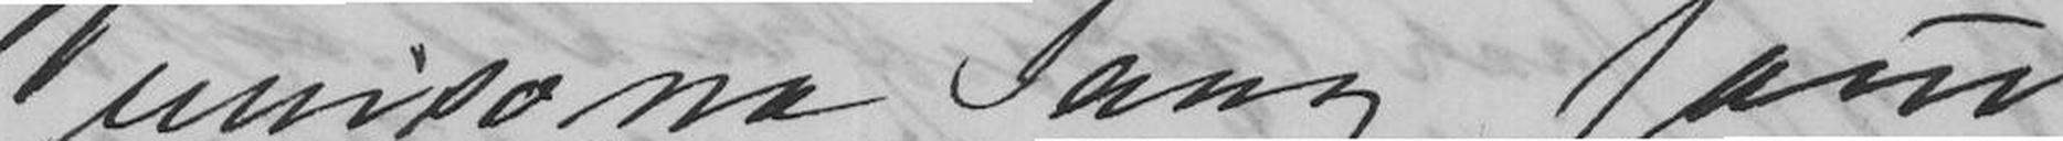unisone Sang som
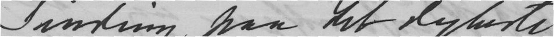Sinding paa det dybeste
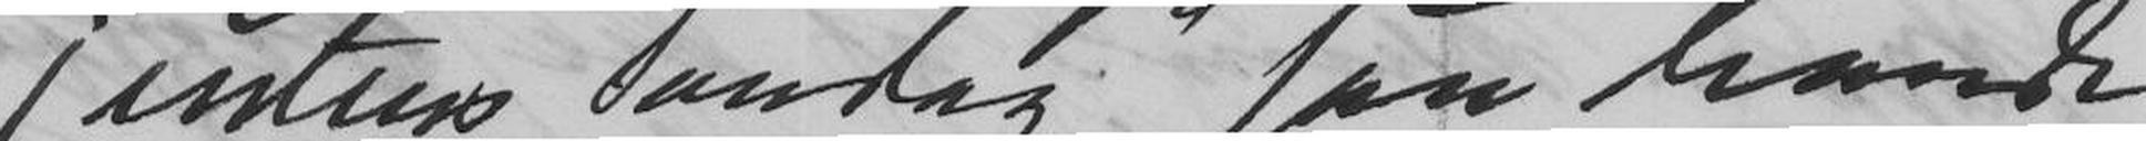jentens Søndag saa havde
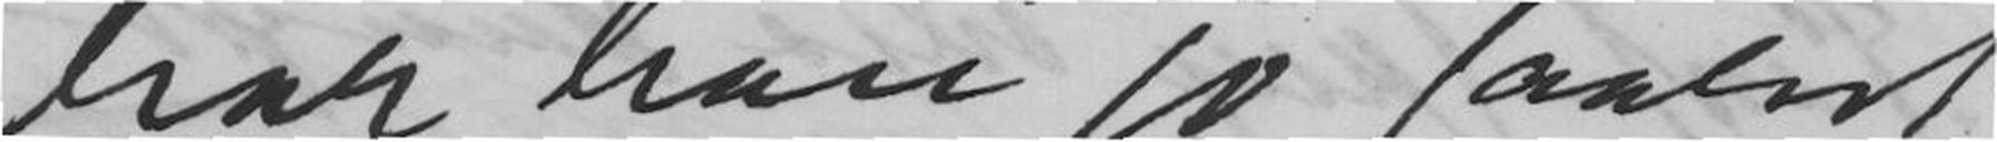har han jo saaret
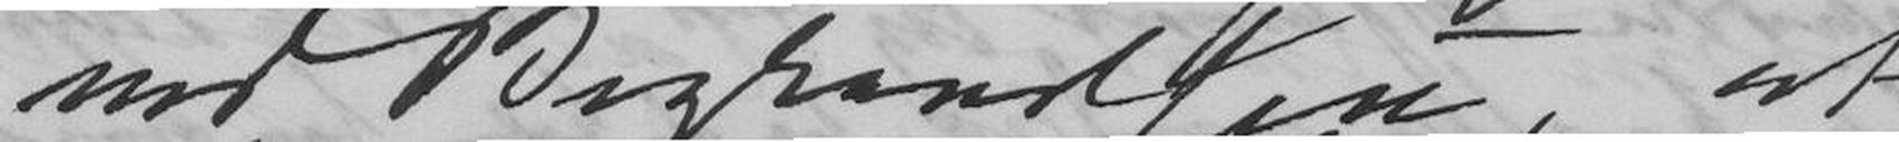ved Begravelsen, at
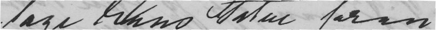lage hans Statue foran
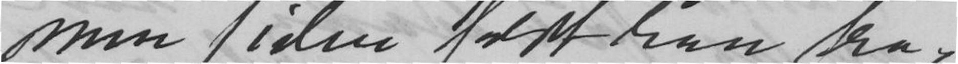men siden falt han fra,
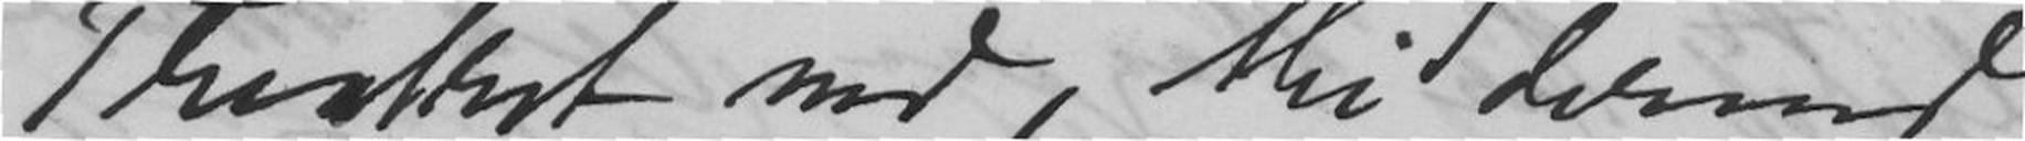Theatret ved, thi derved
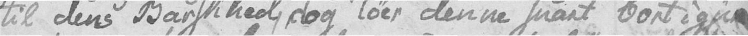til dens Barskhed, dog tøer denne snart bort igjen
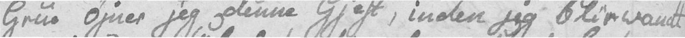Grue øjner jeg denne Gjest, inden jeg blir vandt
